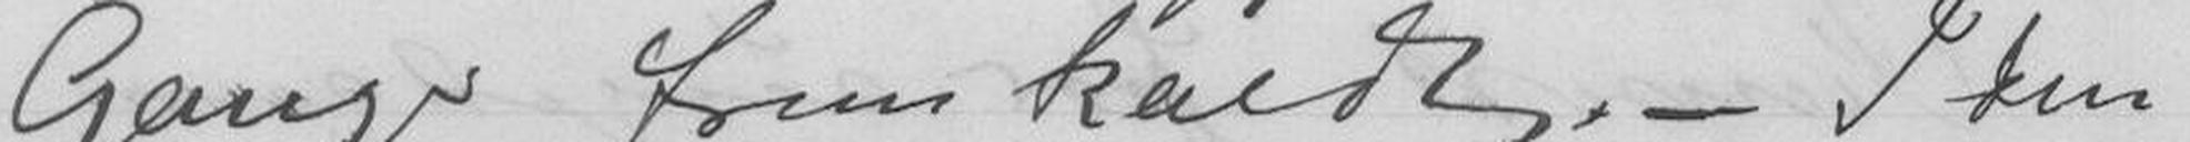Gange fremkaldt, - I den
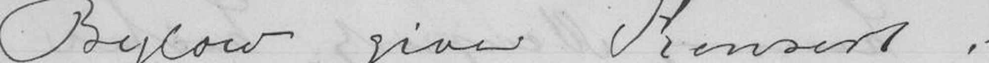Bylow Giver Konsert i
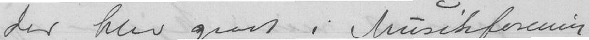der blir givet i Musikforenin-
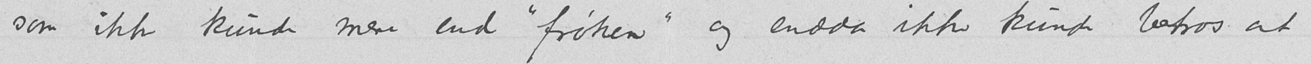som ikke kunde mere end "frøken" og endda ikke kunde betros at
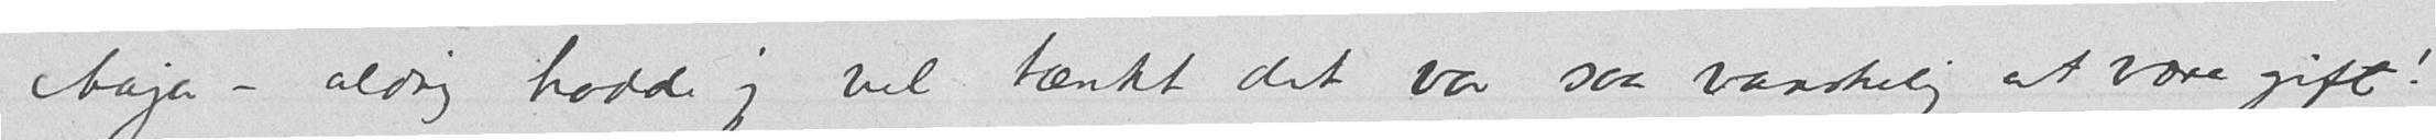Aaja - aldrig hadde jeg vel tænkt det var saa vanskelig at være gift!
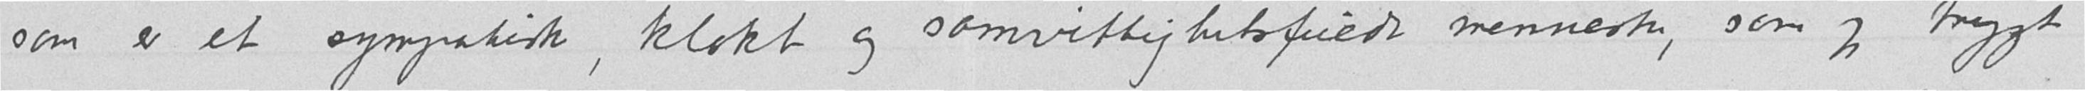som er et sympatisk, klokt og samvittighetsfuldt menneske, som jeg trygt
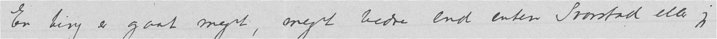En ting er gaaet meget, meget bedre end enten Svarstad eller jeg
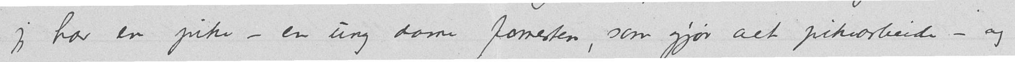jeg har en pike - en ung dame forresten, som gjør alt pikearbeide - og
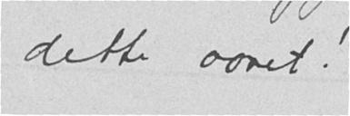dette aaret!
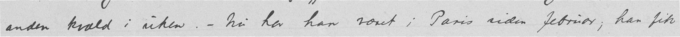anden kvæld i uken. - Nu har han været i Paris siden februar, han fik
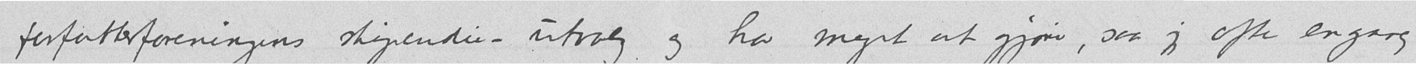forfatterforeningens stipendie-utvalg og har meget at gjøre, saa jeg ofte engang
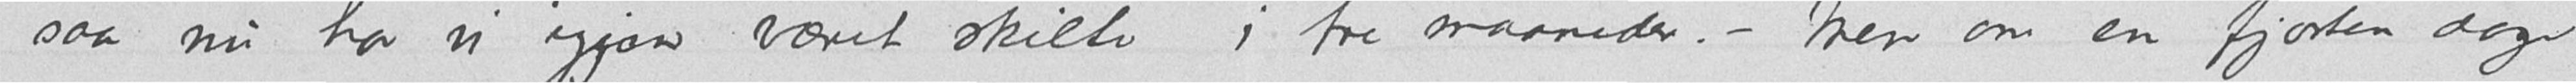saa nu har vi igjen været stille i tre maaneder. - Men om en fjorten dage
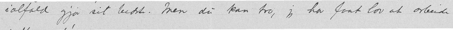ialfald gjør sit bedste. Men du kan tro, jeg ha faat lov at arbeide
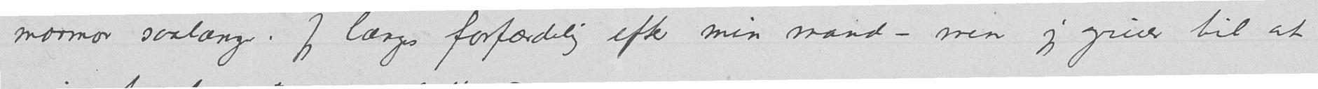mormor saalænge. Jeg længes forfærdelig efter min mand - men jeg gruer til at
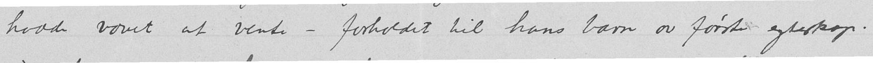hadde vovet at vente - forholdet til hans barn av første ægteskap.
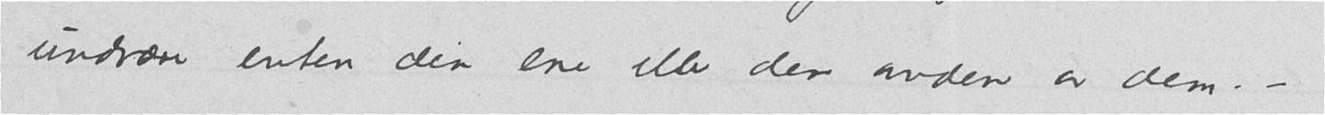undvære enten den ene eller den anden av dem. -
